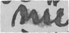me
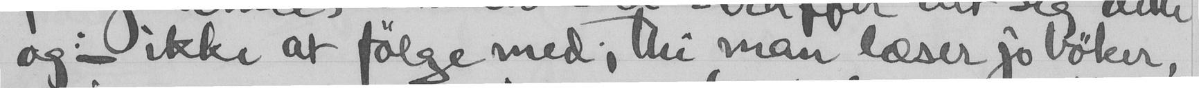og: - ikke at følge med; thi man læser jo bøker,
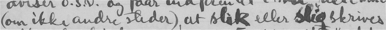(om ikke andre sleder) at slik eller slig skrives
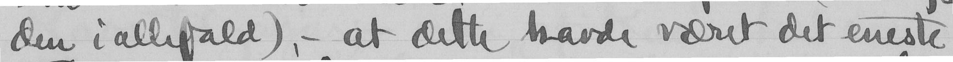den i allefald), - at dette havde været det eneste
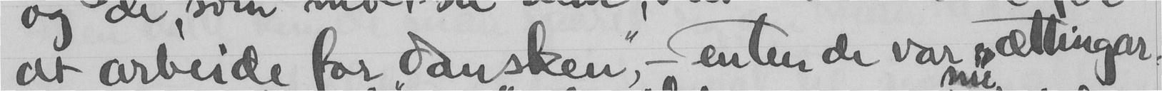at arbeide for "dansken", - enten de var "ættingar"
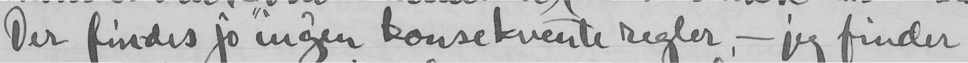Der findes jo ingen konsekvente regler, - jeg finder
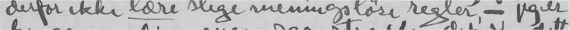derfor ikke lære slige meningsløse regler - jeg er
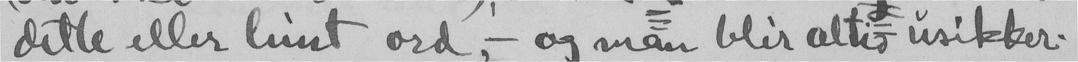dette eller hint ord, - og man blir altid usikker.
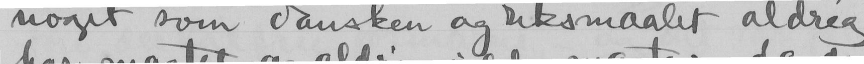noget som dansken og riksmaalet aldrig
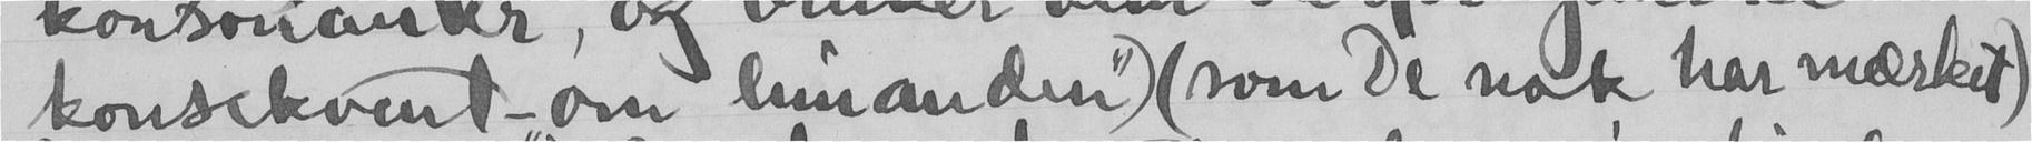konsikvent, "om hinanden") (som De nok har mærket).
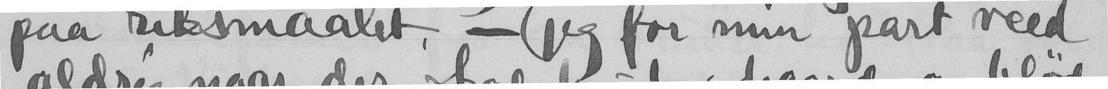paa riksmaalet, - (jeg for min part veed
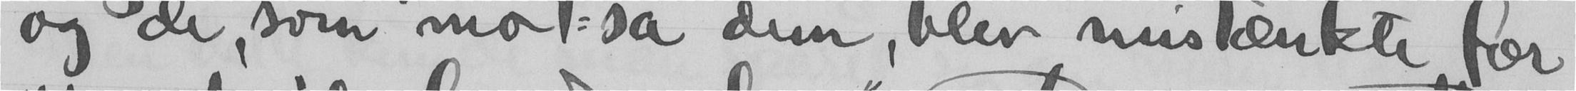og de, som motsa dem, blev mistænkte for
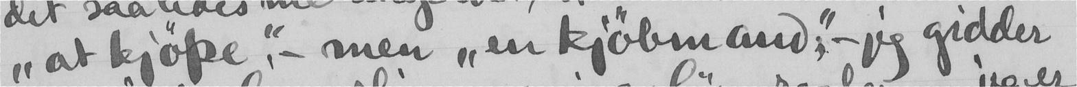"at kjøpe", - men "en kjøbmand"; - jeg gidder
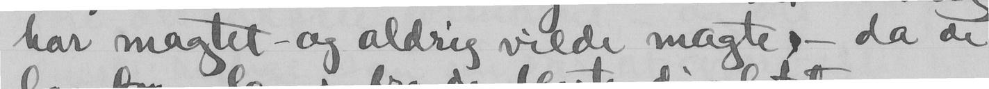har magtet - og aldrig vilde magte, - da de
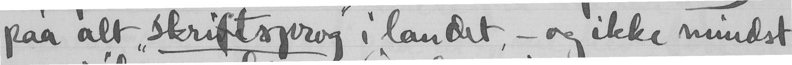paa alt "skriftsprog" i landet", - og ikke mindst
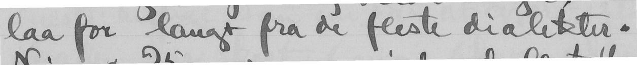laa for langt fra de fleste dialekter.
